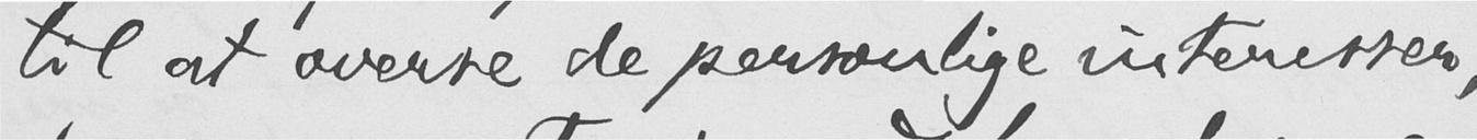til at overse de personlige interesser,
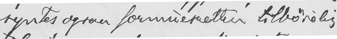syntes ogsaa formuesretten tilbøielig
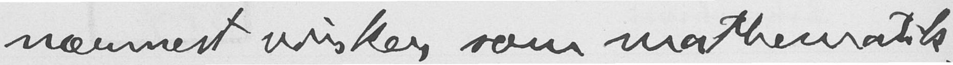nærmest virker som mathematik-
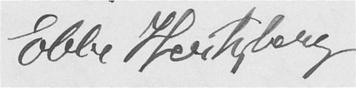Ebbe Hertzberg.
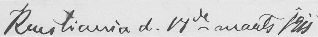Kristiania d. 17de marts 1915.
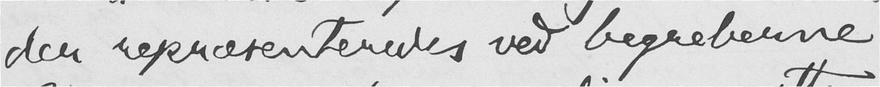der repræsenteredes ved begreberne.
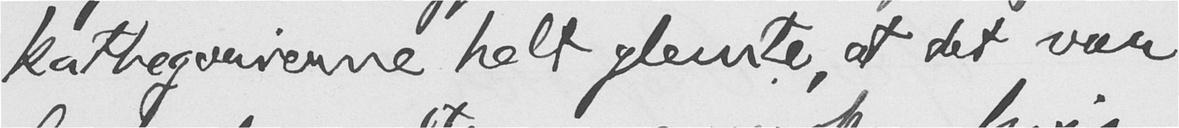kathegorierne helt glemte, at det var
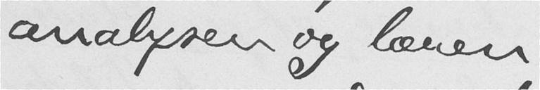analysen og læren.
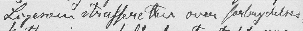Ligesom strafferetten over forbrydelses-
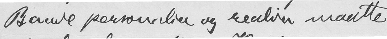Baade personalia og realia maatte
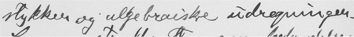stykker og algebraiske udregninger.
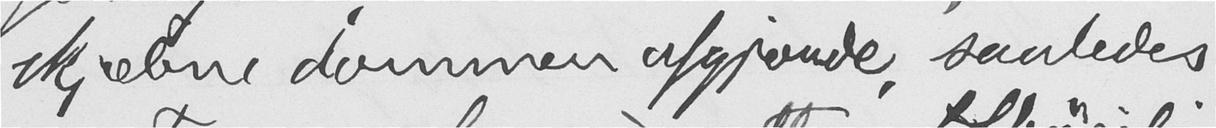skjæbne dommen afgjorde, saaledes
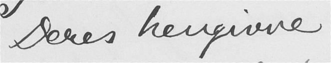Deres hengivne
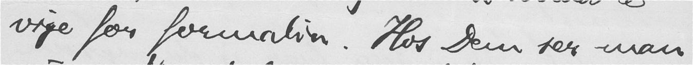vige for formalia. Hos Dem ser man
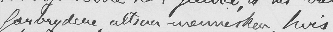forbrydere, altsaa mennesker, hvis
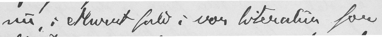nu, i ethvert fald i vor literatur for
